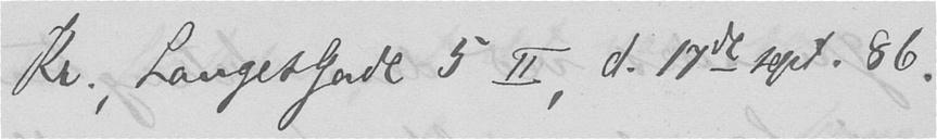Kr. Langesgade 5 II, d. 17de sept. 86.
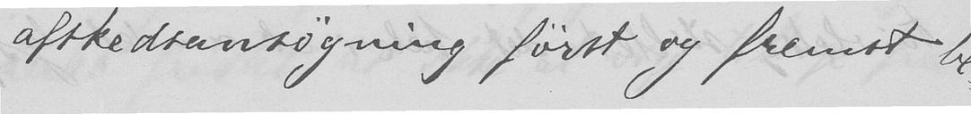afskedsansøgning først og fremst be-
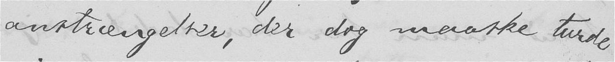anstrængelser, der dog maaske turde
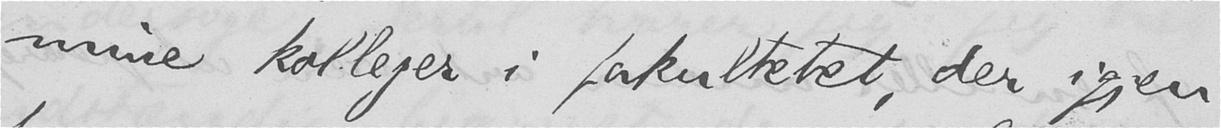mine kolleger i fakultetet, der igjen
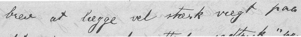brev at lægge vel stærk vægt paa
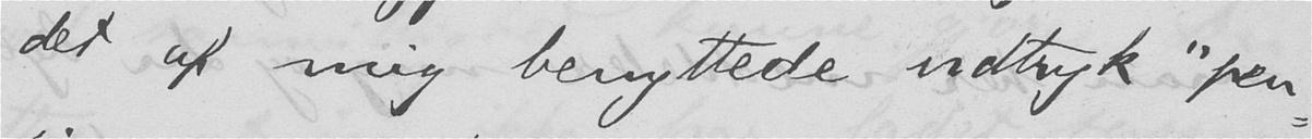det af mig benyttede udtryk "pen-
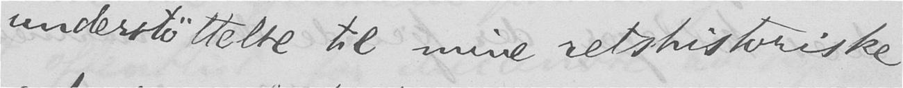understøttelse til mine retshistoriske
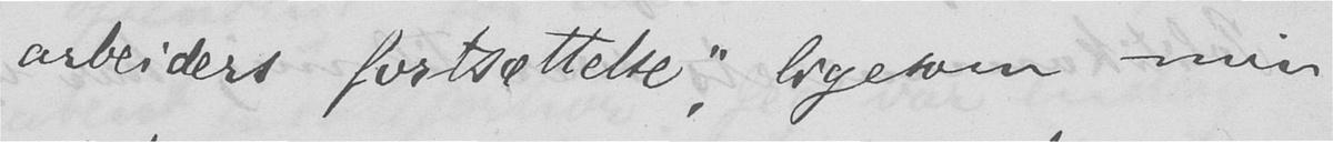arbeiders fortsættelse", ligesom min
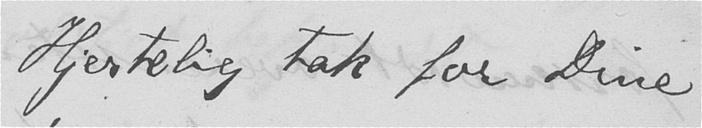Hjertelig tak for Dine
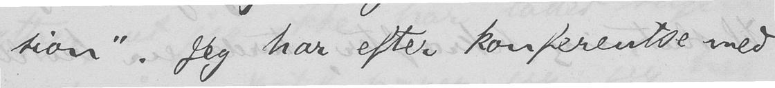sion". Jeg har efter konferentse med
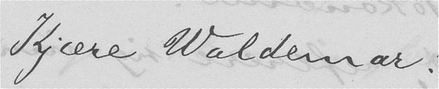Kjære Waldemar!
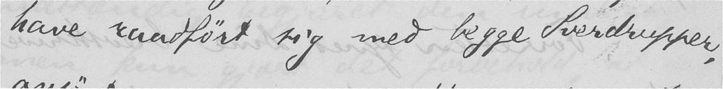have raadført sig med begge Sverdrupper,
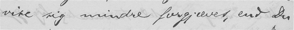vise sig mindre forgjæves, end Du
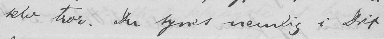selv tror. Du synes nemlig i Dit
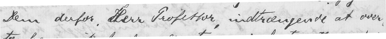Dem derfor, Herr Professor, indtrængende at over-
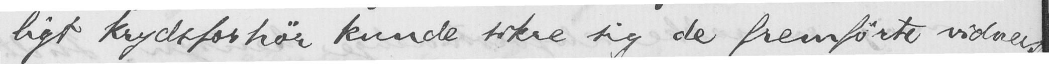ligt krydsforhør kunde sikre sig de fremførte vidners
